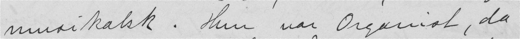musikalsk. Hun var Organist, da
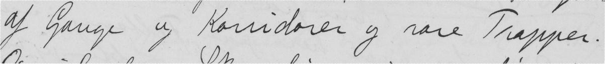af Gange og Korridorer og rare Trapper.
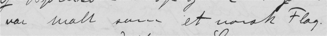var malt som et norsk Flag.
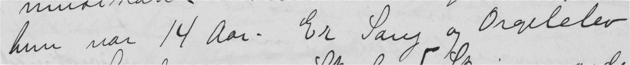hun var 14 Aar. Er Sang og Orgelelev
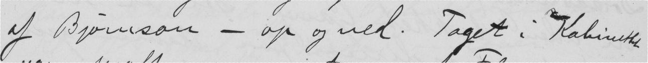af Bjørnson - op og ned. Taget i Kabinettet
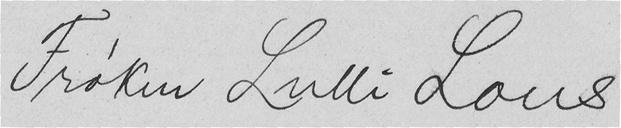Frøken Lulli Lous
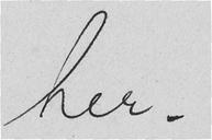her.
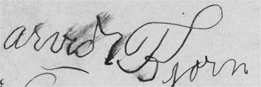Arvid Bjørn
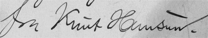fra Knut Hamsun.
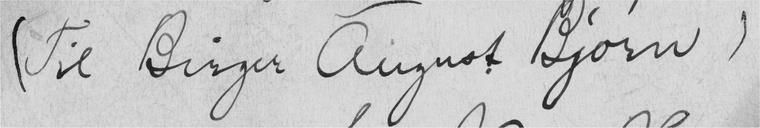(Til Birger August Bjørn)
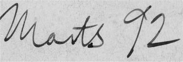Marts 92
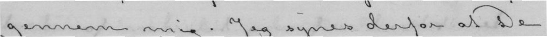gennem mig. Jeg synes derfor at De
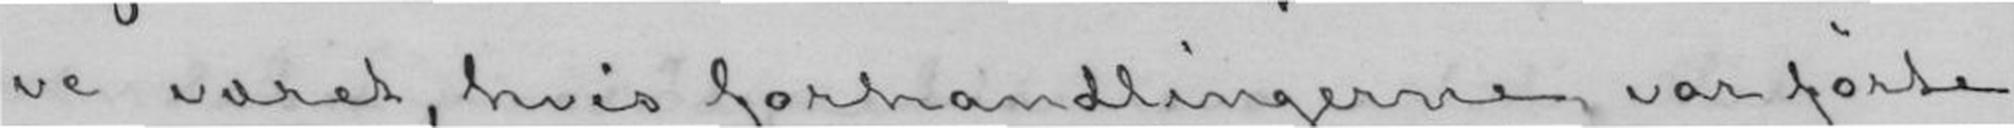ve været, hvis forhandlingerne var førte
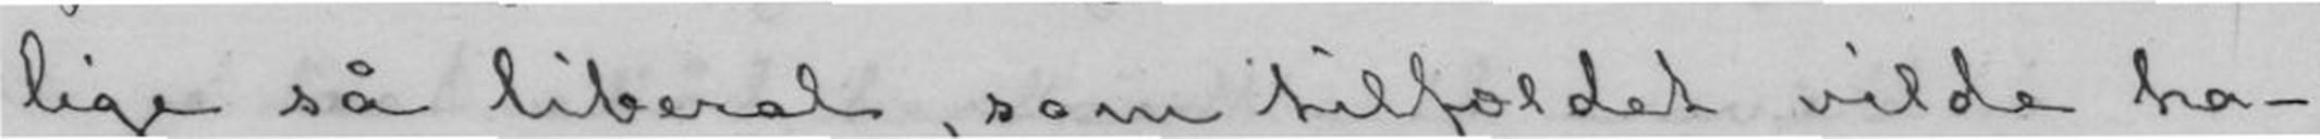lige så liberal, som tilfældet vilde ha-
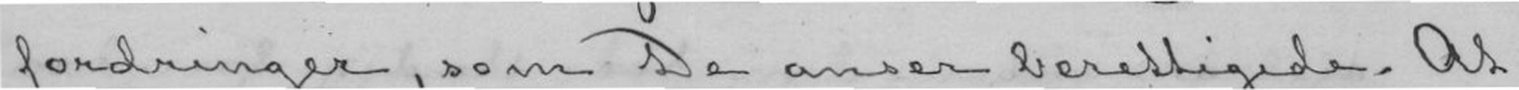fordringer, som De anser berettigede. At
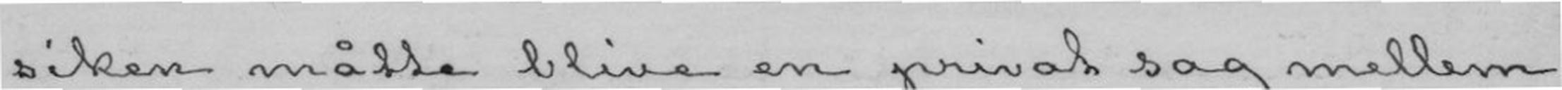siken måtte blive en privat sag mellem
